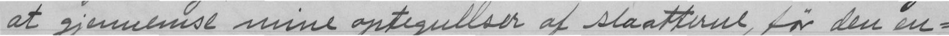at gjennemse mine optegnellser af slaatterne, før den en-
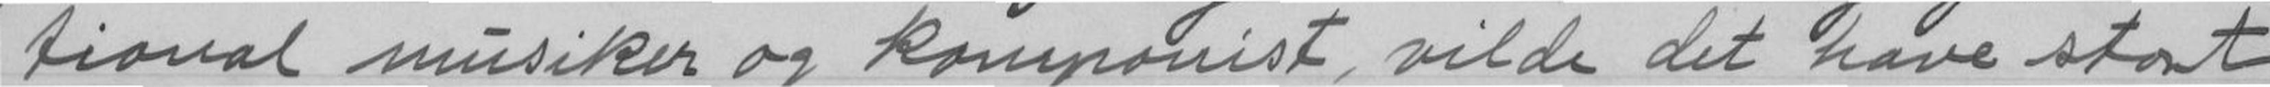tional musiker og komponist, vilde det have stort
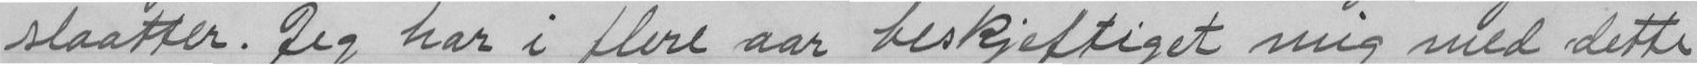slaatter. Jeg har i flere aar beskjeftiget mig med dette
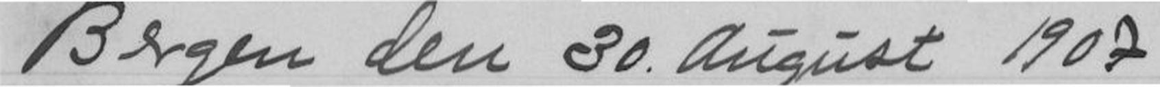Bergen den 30. august 1907
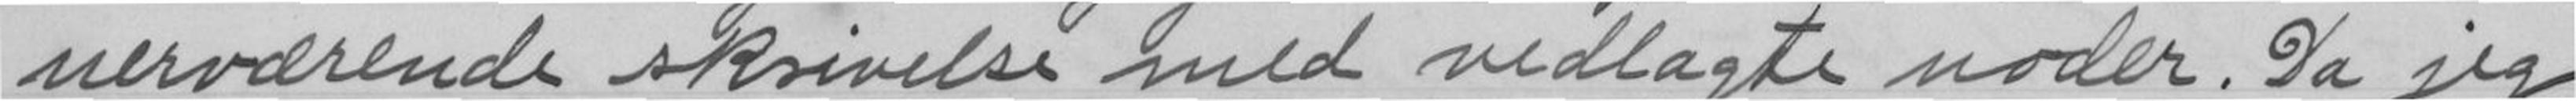nerværende skrivelse med vedlagte noder. Da jeg
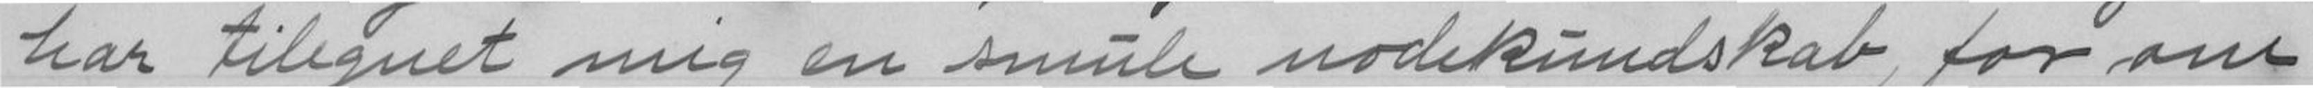har tilegnet mig en smuk nodekunskab for om
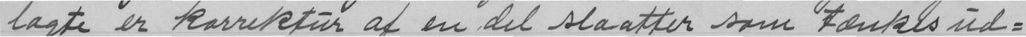lagte er korrektur af en del slaatter som tænkes ud-
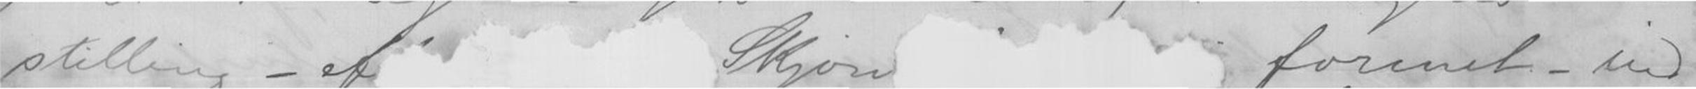stillingen - ef Skjøn forenet - ind
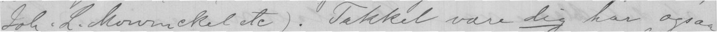Joh. Mowinckel etc). Takket være dig har ogsaa
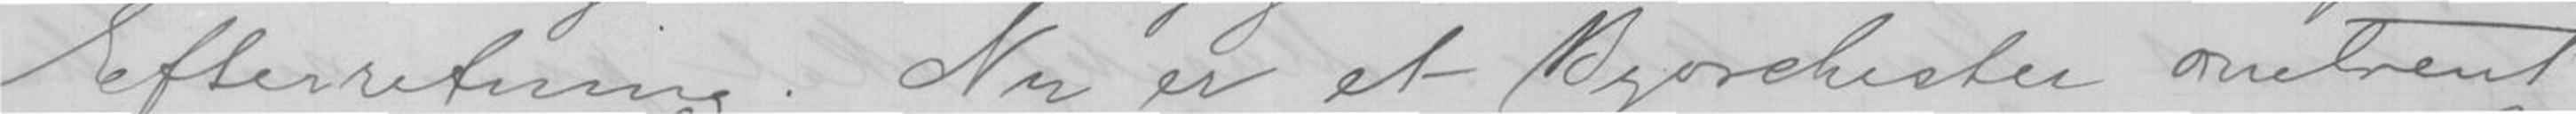Efterretning. Nu er et Byorchester omtrent
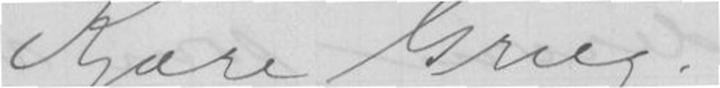Kjære Grieg!
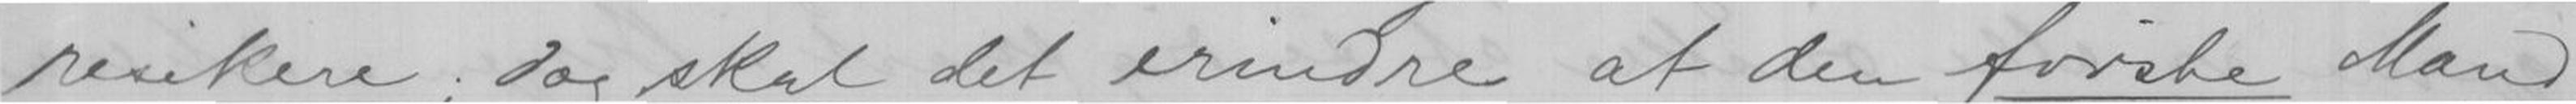risikere; dog skal det erindre at den første Mand
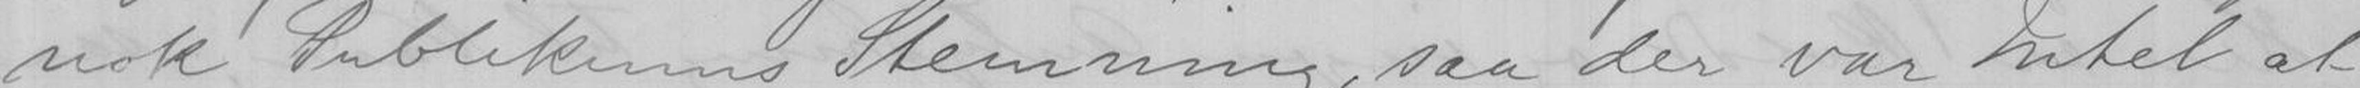nok Publikums Stemning, saa der var Intet at
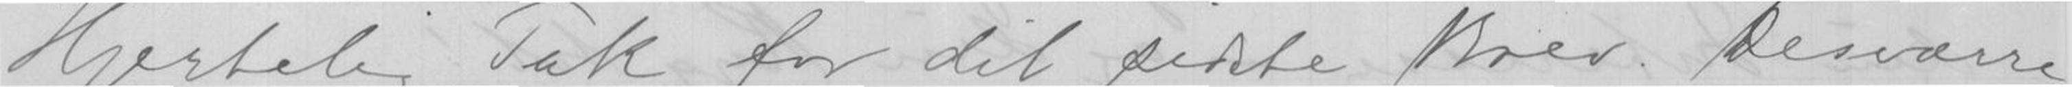Hjertelig Tak for dit sidste Brev. Desværre
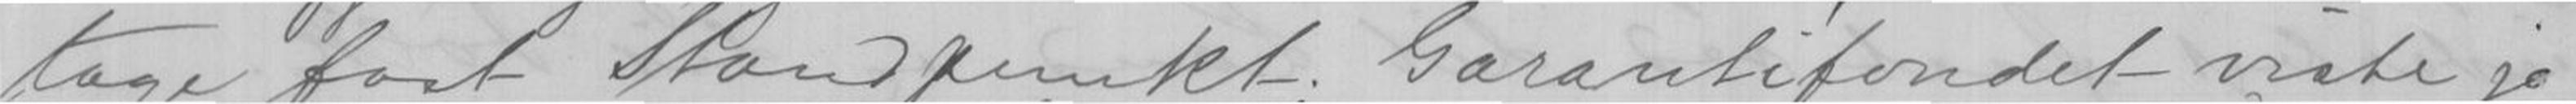tage fort Standpunkt, Garantifondet viste jo
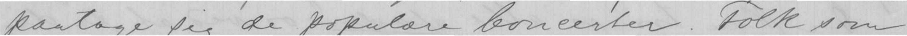paatage sig de populære Concerter. Folk som
